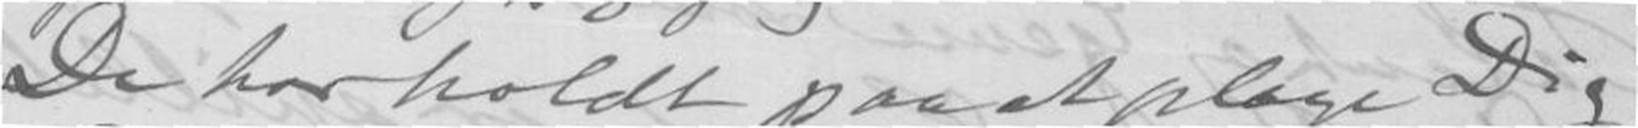De har holdt paa at plage Dig
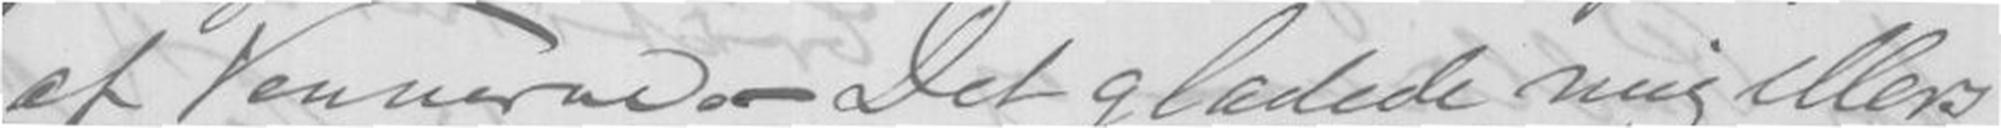af Vennerne. Det glædede mig ellers
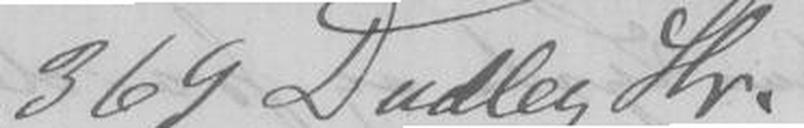369 Dudley Str.
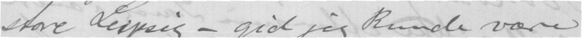store Leipzig - gid jeg kunde være
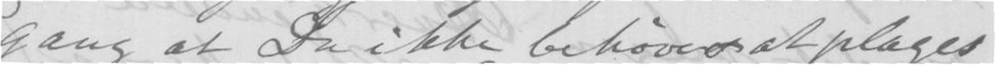gang at Du ikke behøves at plages
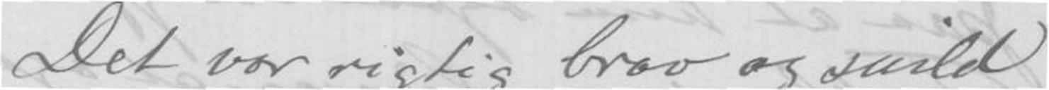Det var rigtig brav og snild
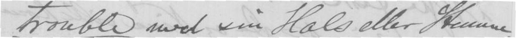trouble med sin Hals eller Stemme,
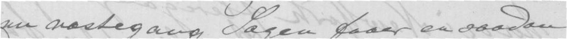nu næstegang Sangen faaer en saadan
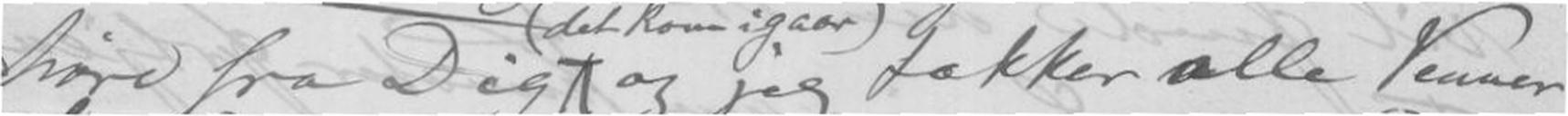høre fra Dig og jeg takker alle Venner
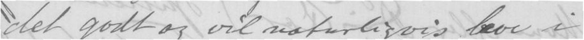det godt og vil naturligvis leve i
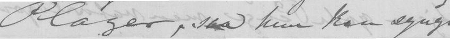Plager, saa hun kan synge
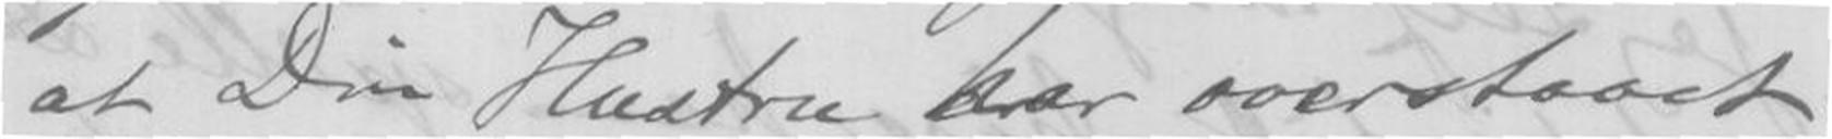at Din Hustru har overstaaet
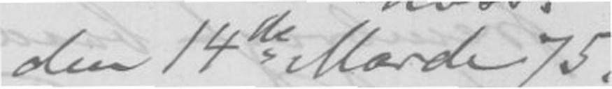den 14de Marde 75.
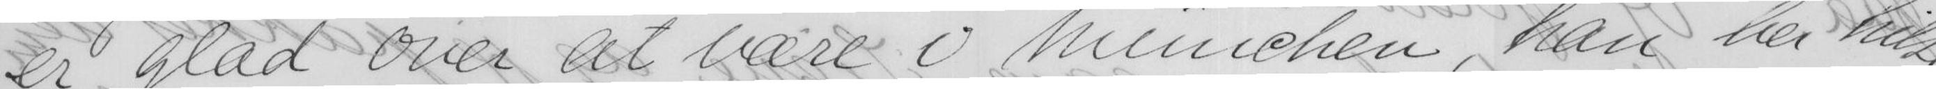er glad over at være i Munchen, han ber hilse
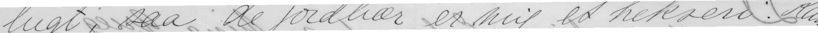lugt, saa de jordbær er mig et hekseri. Kanske
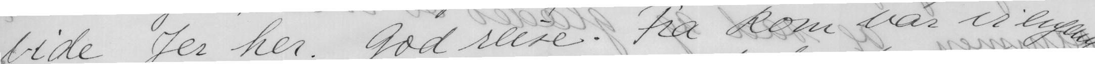vide Jer her. God reise. Fra Rom var vi engang
